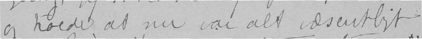og troede, at nu var alt væsentligt
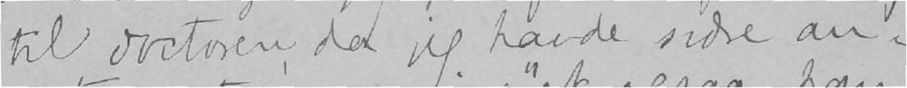til doctoren, da jeg havde svære an-
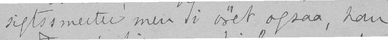sigtssmerter men i øret ogsaa, han
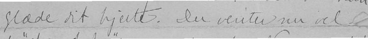glæde dit hjerte. Du venter nu vel
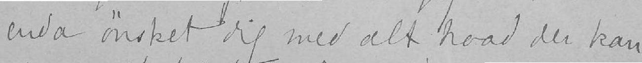enda ønsket dig med alt, hvad der kan
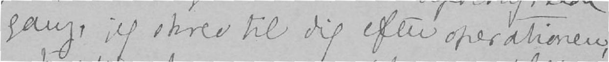gang, jeg skrev til dig efter operationen,
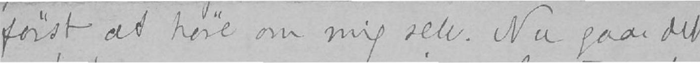først at høre om mig selv. Nu gaar det
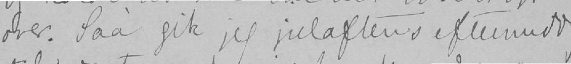over. Saa gik jeg julaftens eftermiddag
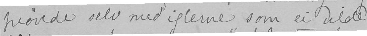prøvede selv med iglerne som ei vilde
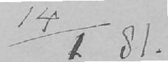14/1 81.
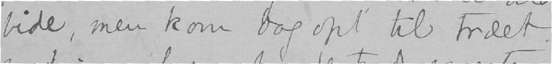bide, men kom dog opt til træet
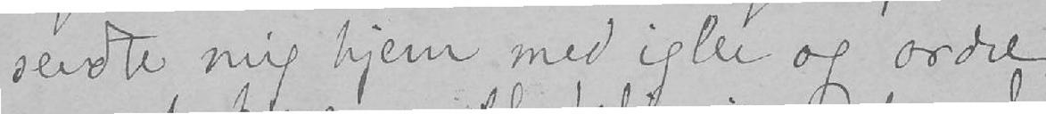sendte mig hjem med igler og ordre
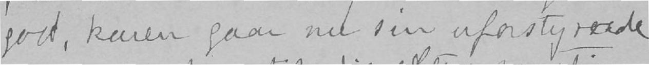godt, kuren gaar nu sin uforstyrrede
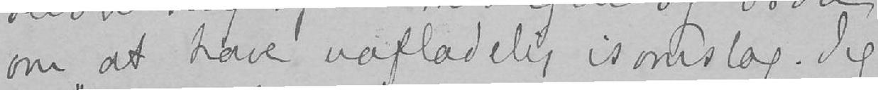om at have uafladelig isomslag. Jeg
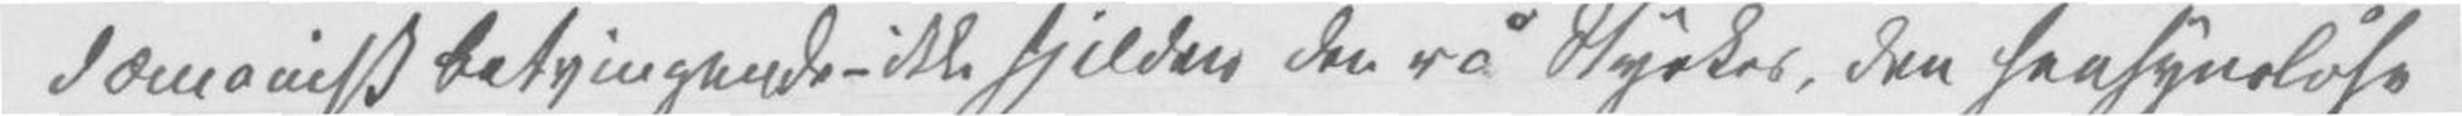dæmonisk betvingende – ikke sjelden den rå Styrkes, den hensynsløse
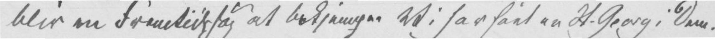blir en Fremtidssag at bekjempe. Vi har fået en St. Georg i Dem.
